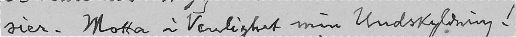sier. Motta i Venlighet min Undskyldning!
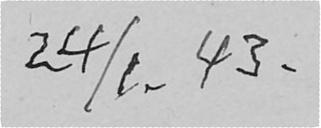24/1. 43.
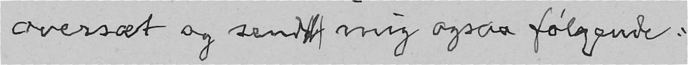oversæt og sendt mig ogsaa følgende:
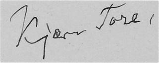Kjære Tore,
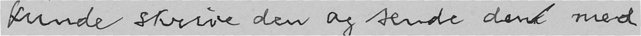kunde Skrive den og sende den med
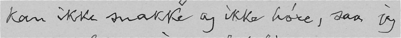kan ikke snakke og ikke høre, saa jeg
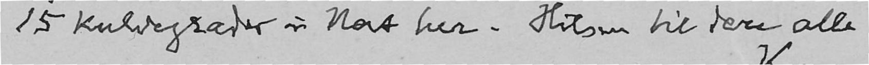15 Kuldegrader i Nat her. Hilsen til dere alle
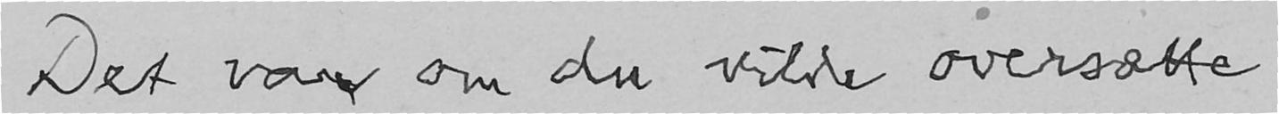Det var om du vilde oversætte
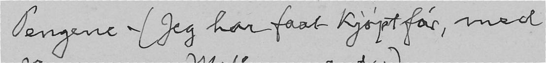Pengene - (Jeg har faat kjøpt før, med
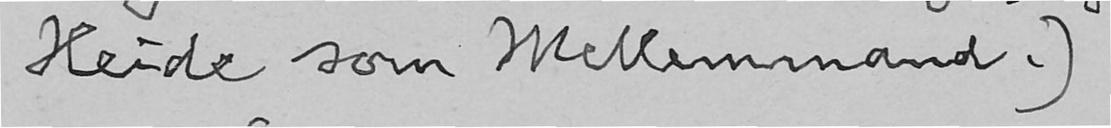Heide som Mellemmand.)
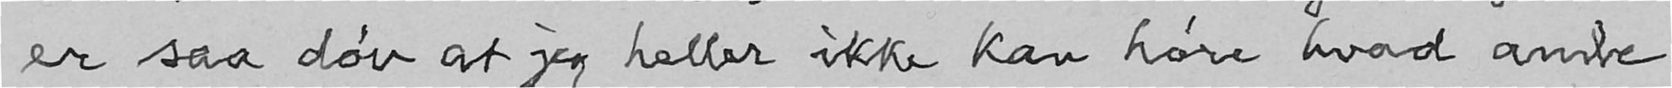er saa døv at jeg heller ikke kan høre hvad andre
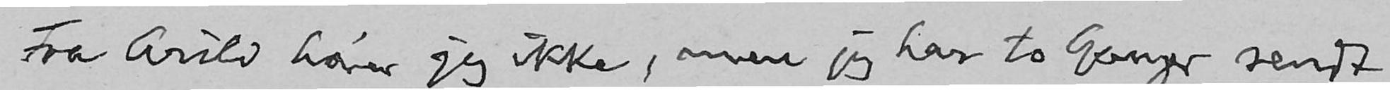Fra Arild hører jeg ikke, men jeg har to Ganger sendt
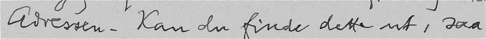Adressen. Kan du finde dette ut, saa
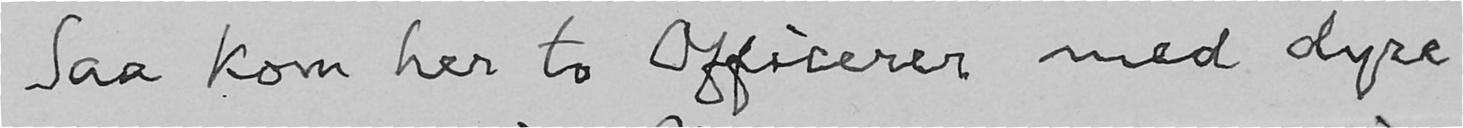Saa kom her to Officerer med dyre
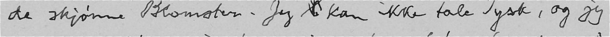de skjønne Blomster. Jeg f kan ikke tale Tysk, og jeg
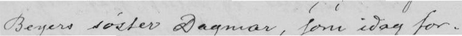Beyers søster Dagmar, som idag for-
